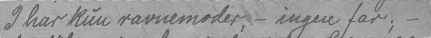I har kun ravnemoder, - ingen far; -
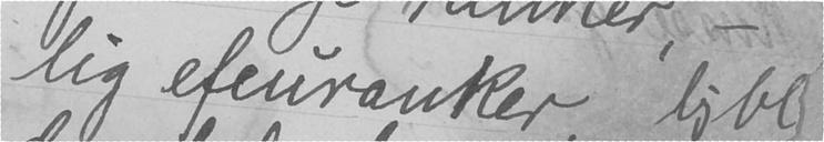lig efeuranker, ljbl
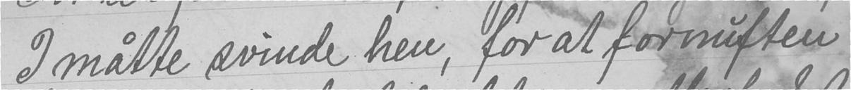I måtte svinde hen, for at fornuften
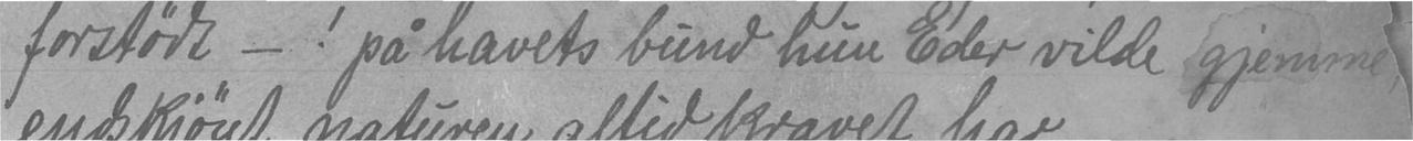forstødt - ! på havets bund hun Eder vilde gjemme,
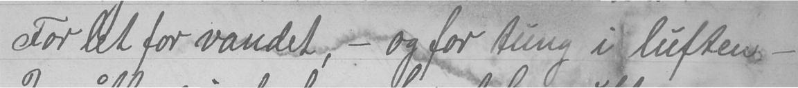For let for vandet, - og for tung i luften -
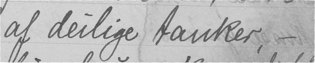af deilige tanker, -
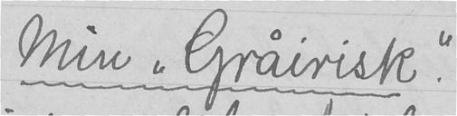Min "Gråirisk."
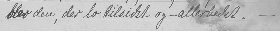blev den, der lo tilsidst og - allerbedst. -
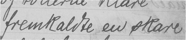fremkaldte en skare
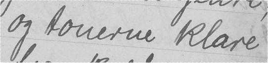og tonerne klare
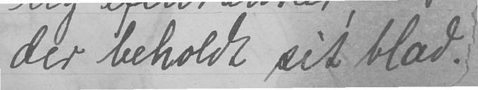der beholdt sit' blad.
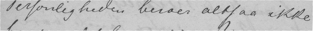Personligheden beroer altsaa ikke
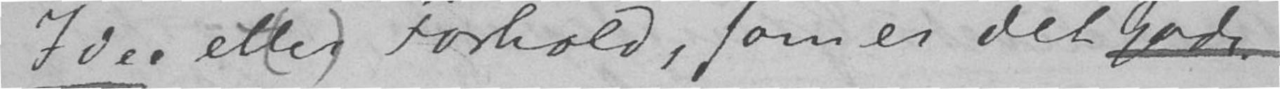Idee eller Forhold, som er det Gode.
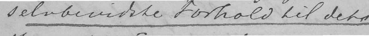selvbevidste Forhold til dets
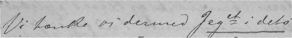Vi tænke nu dermed Jeget i dets
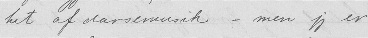tet af dansemusik - men jeg er
